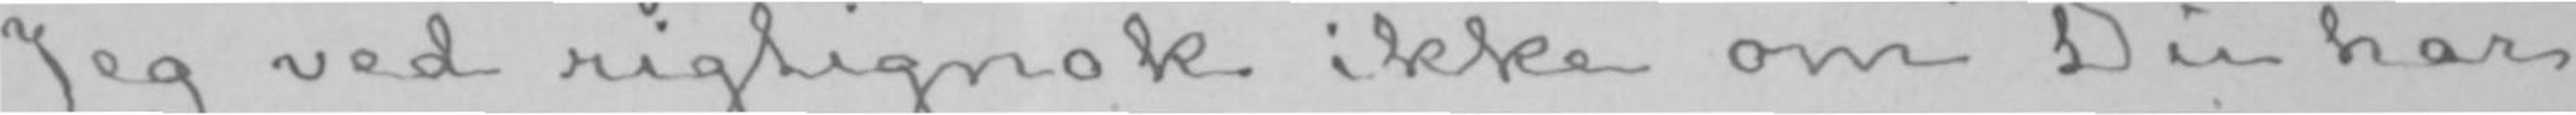Jeg ved rigtignok ikke om Du har
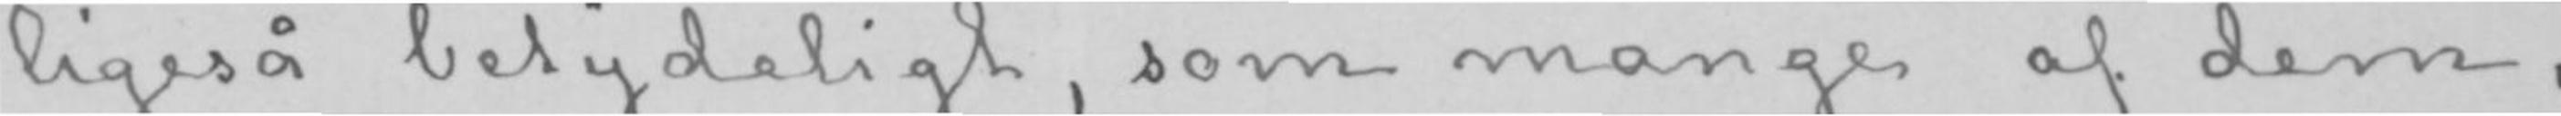ligeså betydeligt, som mange af dem,
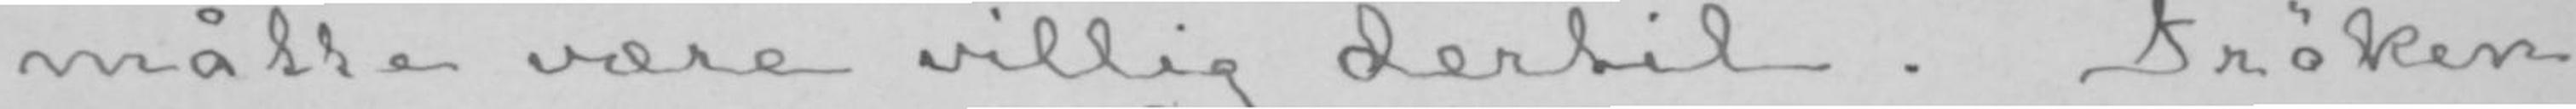måtte være villig dertil. Frøken
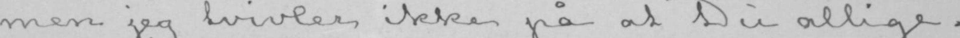men jeg tvivler ikke på at Du allige-
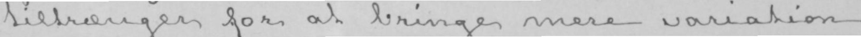tiltrænger for at bringe mere variation
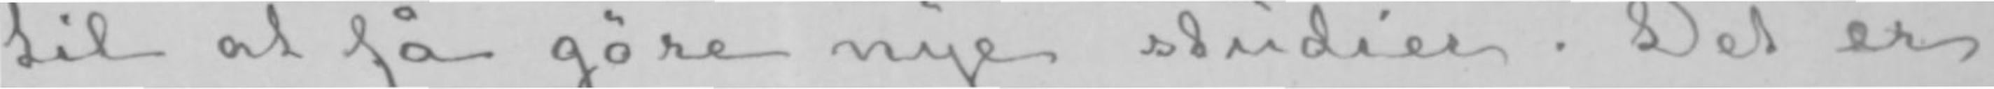til at få gøre nye studier. Det er
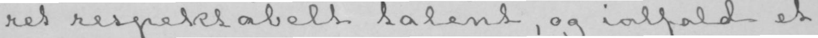ret respektabelt talent, og ialfald et
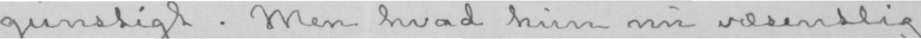gunstigt. Men hvad hun nu væsentlig
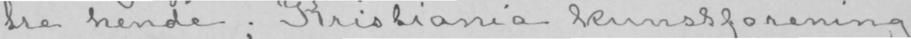tre hende; Kristiania kunstforening
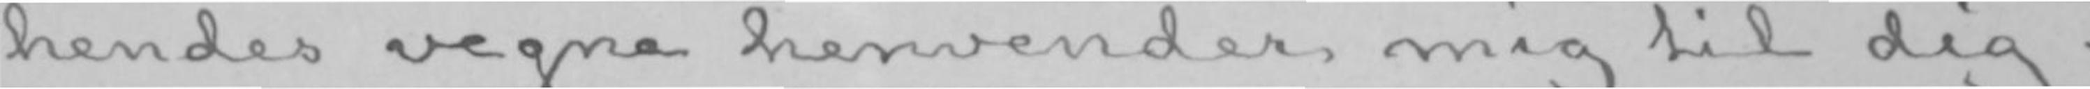hendes vegne henvender mig til dig.
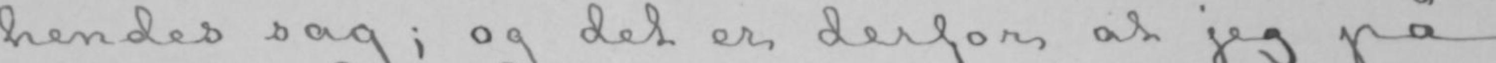hendes sag; og det er derfor at jeg på
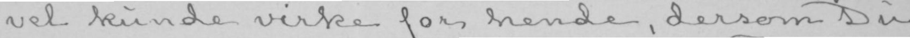vel kunde virke for hende, dersom Du
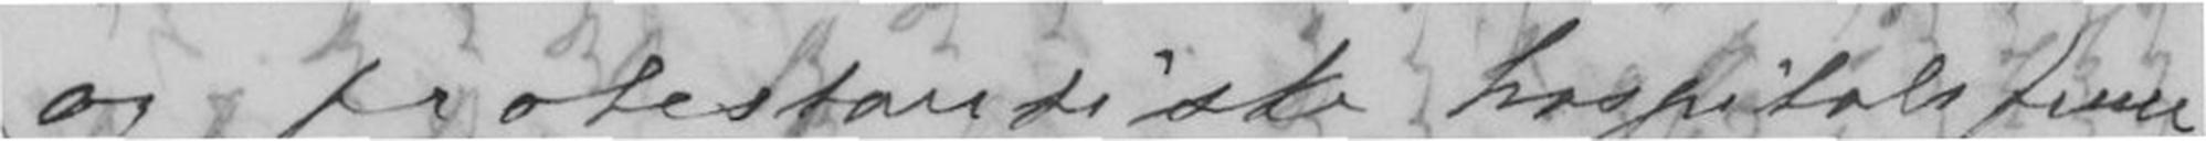og protestantiske hospitals fruer
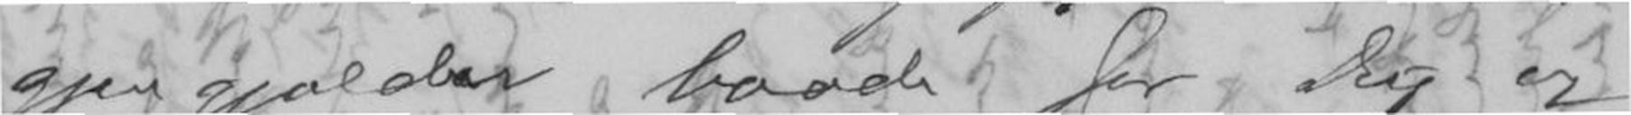gjengjelder baade for Dig og
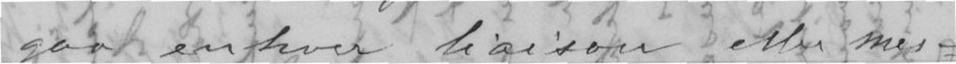gaa enhver liaison eller mes-
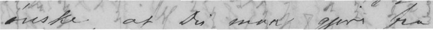ønske, at Du maa gjøre fra
